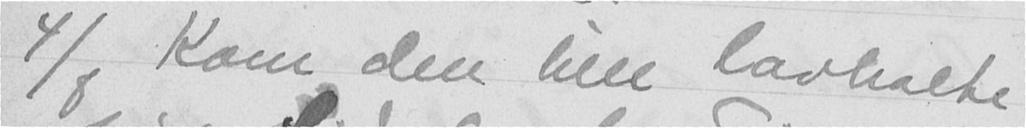4/8 Kom den lille lavhalte
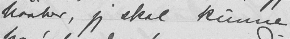haaber, jeg skal kunne
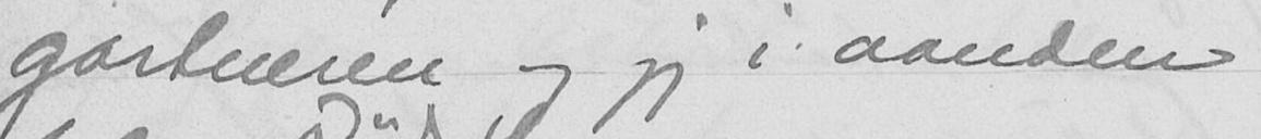gartneren og jeg i aanden
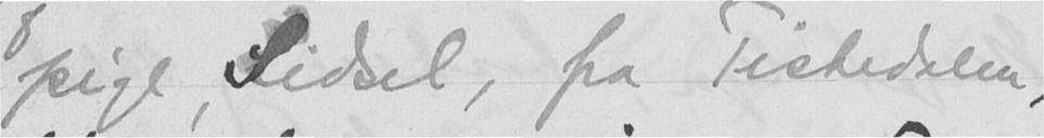pige, Sidsel, fra Tistedalen,
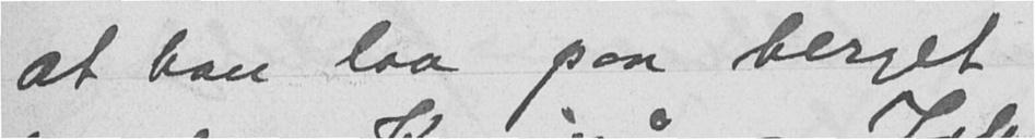at han laa paa berget
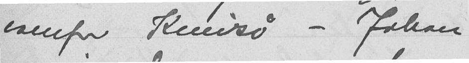ovenfor Knivsø - Johan
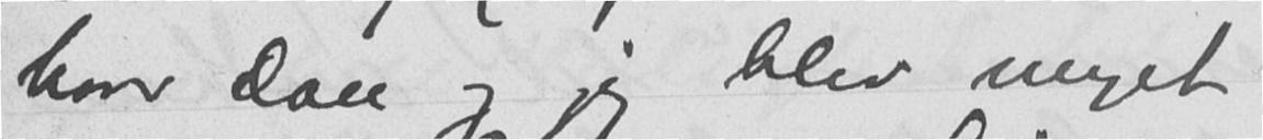hvor Don og jeg blev meget
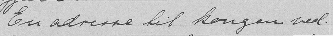En adresse til kongen ved-
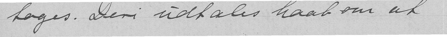toges. Deri udtales haab om at
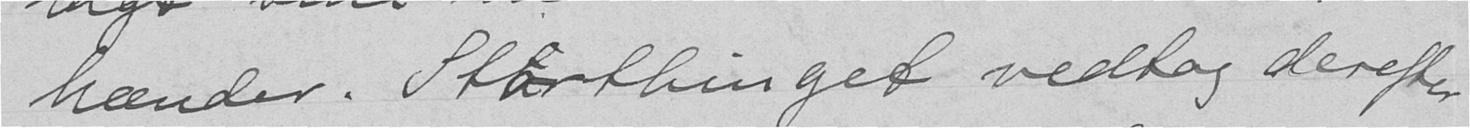hænder. Storthinget vedtog derefter
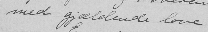med gjældende love.
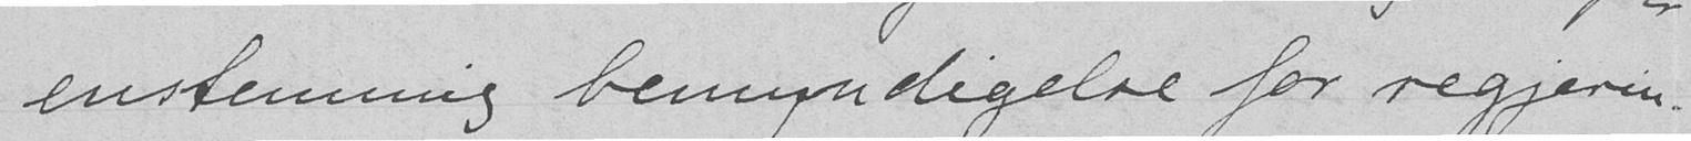enstemmig bemyndigelse for regjerin-
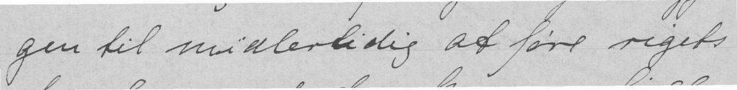gen til midlertidig at føre rigets
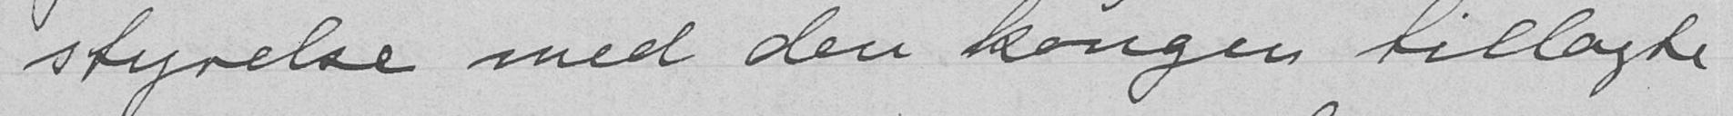styrelse med den kongen tillagte
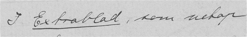I Extrablad, som netop
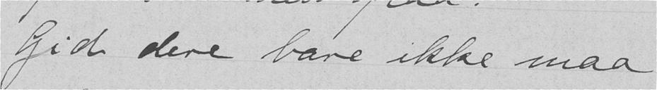Gid dere bare ikke maa
